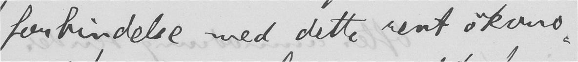forbindelse med dette rent økono-
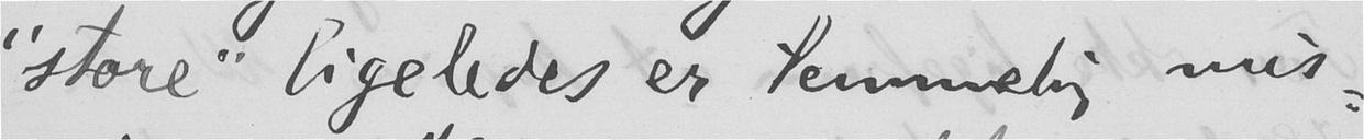"store" ligeledes er temmelig mis-
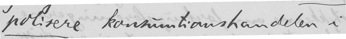polisere konsumtionshandelen i
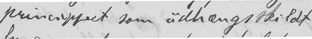princippet som udhængsskildt
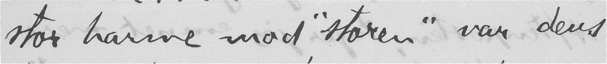stor harme mod "storen", var dens
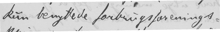kun benyttede forbrugsforenings-
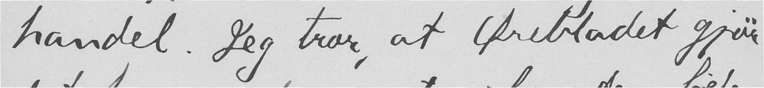handel. Jeg tror, at Ørebladet gjør
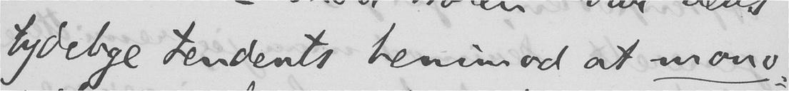tydelige tendents henimod at mono-
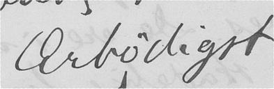Ærbødigst
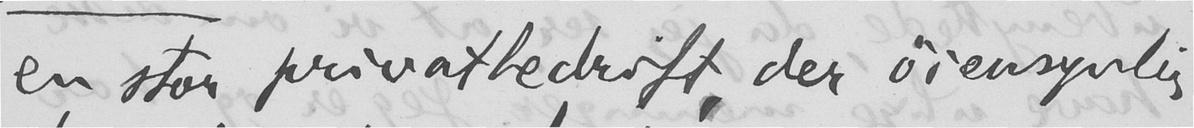en stor privatbedrift, der øiensynlig
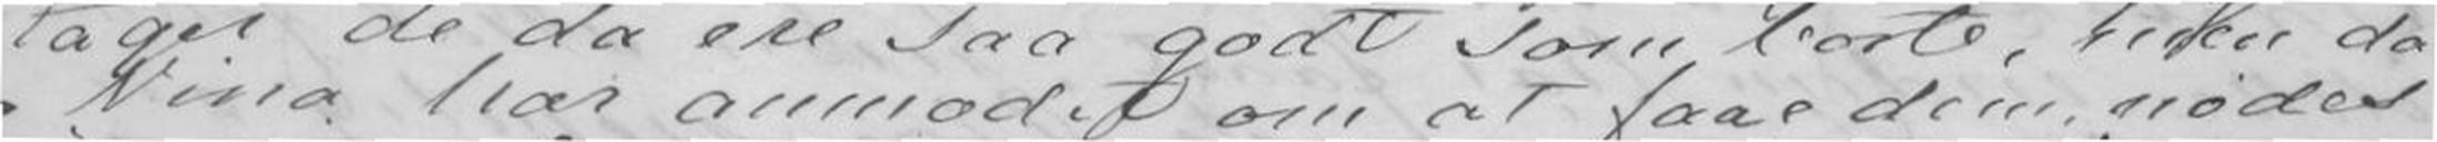Nina har anmodet om at faae dem, nødes
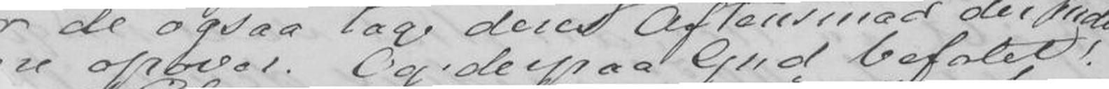re opover. Og derpaa Gud befalet!
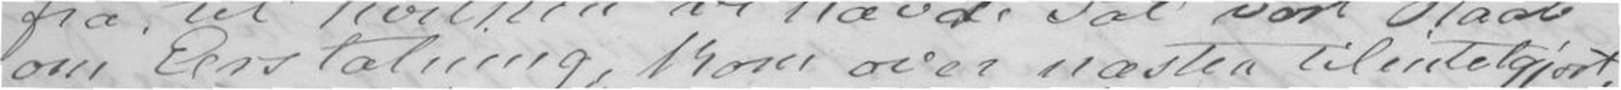om Erstatning kom over næsten tilintetgjort,
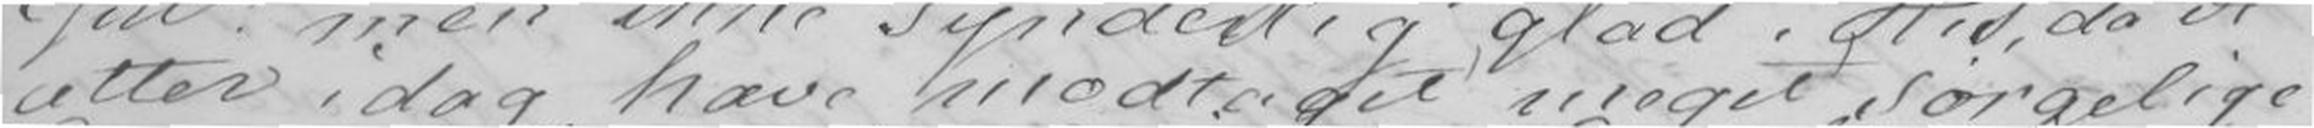atter idag have modtaget meget sørgelige
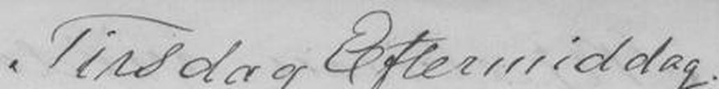Tirsdag Eftermiddag.
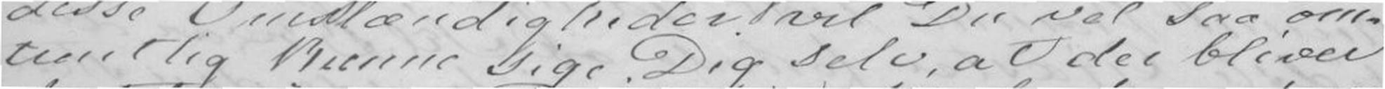trentlig kunne sige Dig selv at der bliver
